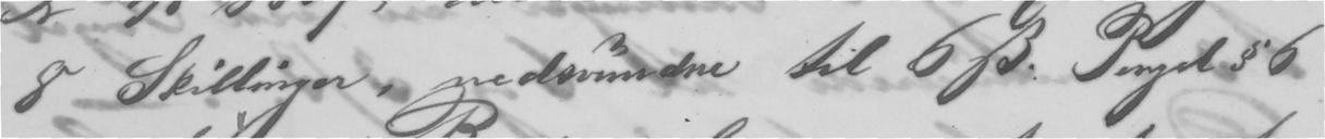8 Skillinger, nedsvundne til 6 ẞ. Pengel §6
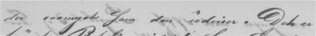der saameget Som den udviser. Det er
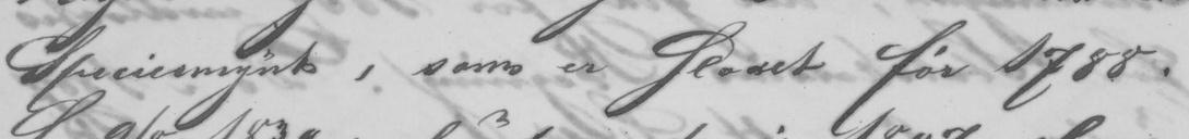Speciesmynt, som Paaset før 1788.
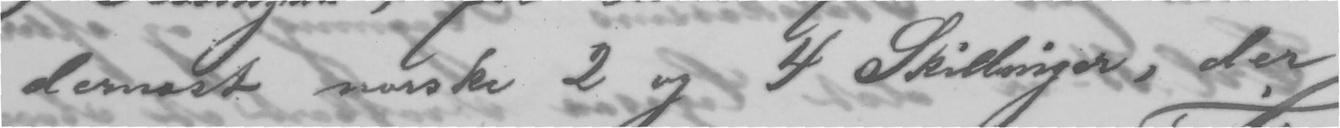dernest norske 2 og 4 Skillinger, der
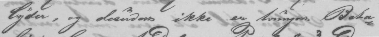lyder, og desuden ikke en tvungen Beta-
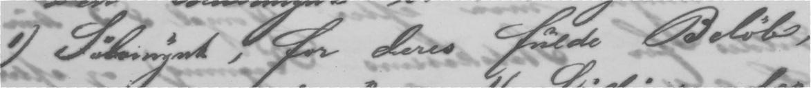1) Sølvmynt, for deres fulde Beløb,
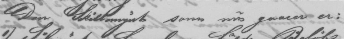Den Skillemynt som nu gaaeer er:
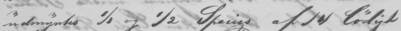udmyntes ¹⁄₁ og ½ Species af 14 løligt
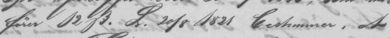fører 12 ẞ. L. 20/8 1821 bestemmer, at
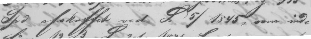Spd afskaffet ved L 5/1845, som ind-
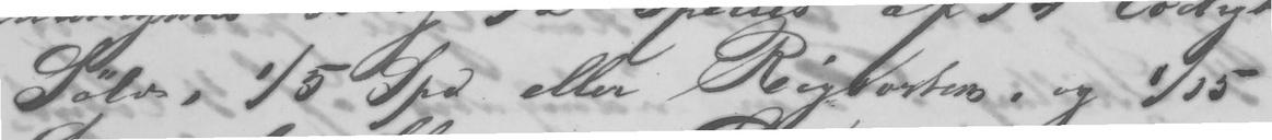Sølv, ¹⁄₅ Spd eller Rigsorten, og ¹⁄₁₅
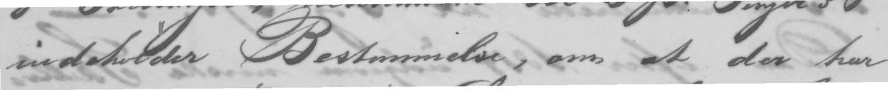indeholder Bestemmelse, om at der har
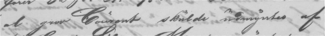al grov Courant skulde udmyntes af
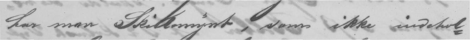har man Skillemynt, som ikke indehol-
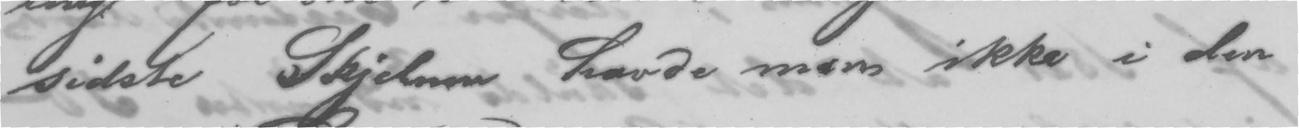sidste Skjelnen havde man ikke i den
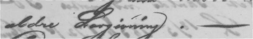ældre Lovgivning. -
